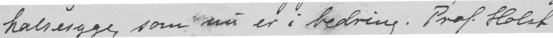halsesyge, som nu er i bedring. Prof. Holst
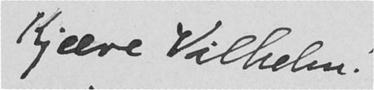Kjære Vilhelm!
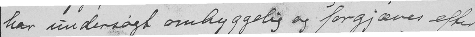har undersøgt omhyggelig og forgjæves efter
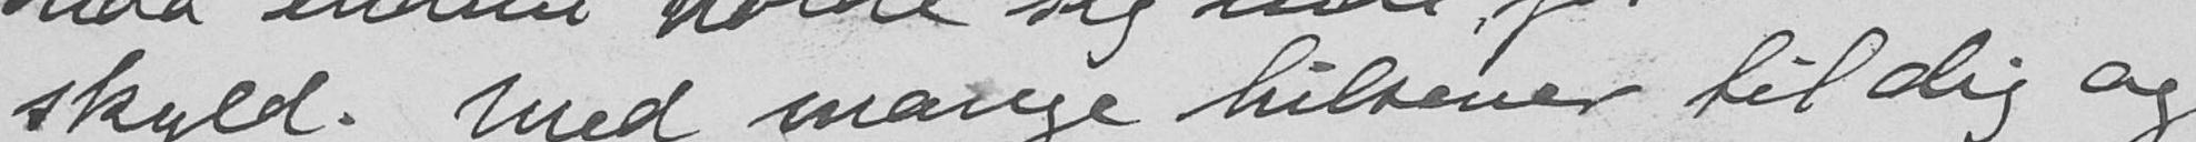skyld. Med mange hilsener til dig og
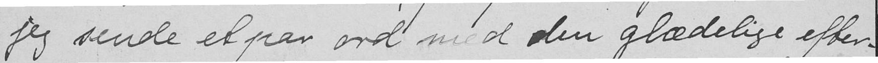jeg sende et par ord med den glædelige efter-
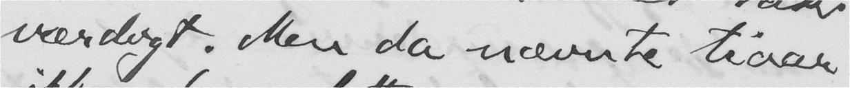værdigt. Men da nævnte tiaar
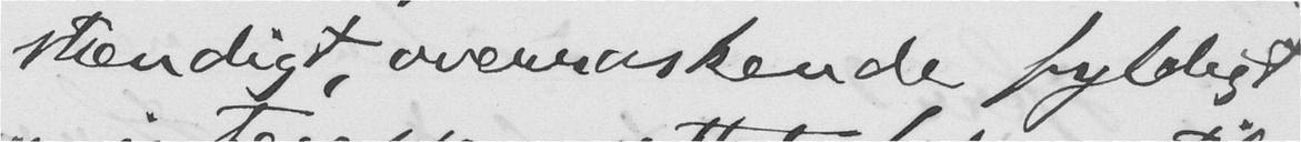stændigt, overraskende fyldigt
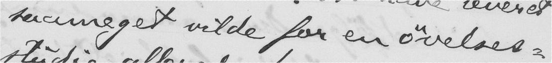saameget vilde for en øvelses-
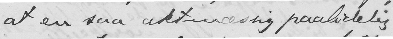at en saa aktmæssig paalidelig
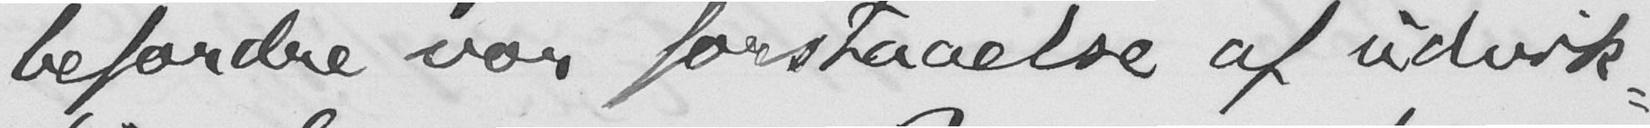befordre vor forstaaelse af udvik-
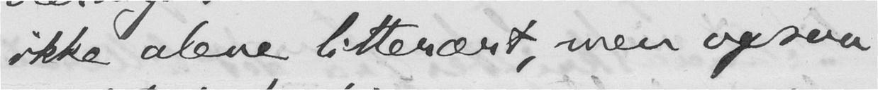ikke alene litterært, men ogsaa
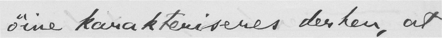øine karakteriseres derhen, at
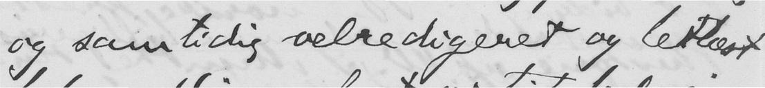og samtidig velredigeret og letlæst
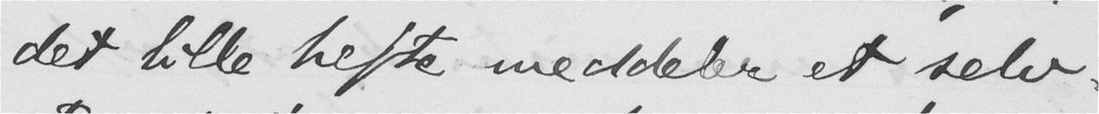det lille hefte meddeler et selv-
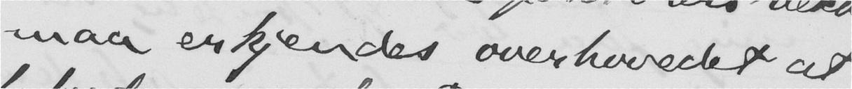maa erkjendes overhovedet at
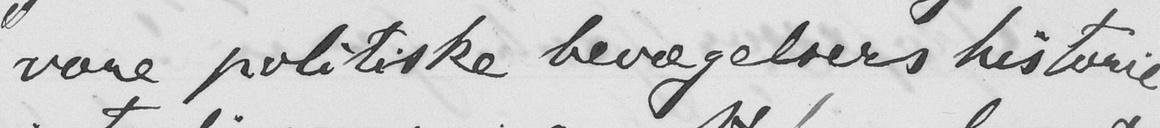vore politiske bevægelsers historie
